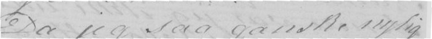Da jeg saa ganske nylig
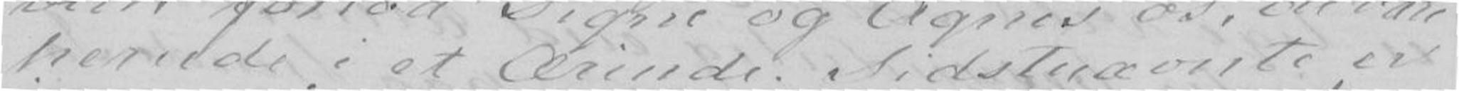herude i et rask Ærinde. Sidstnævnte er
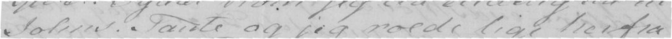Johns. Tante og jeg roede lige herfra
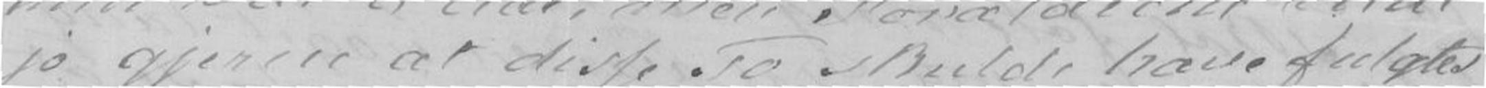jo gjerne at disse To skulde have fulgtes
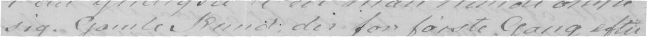sig. Gamle Knud der for første Gang efter
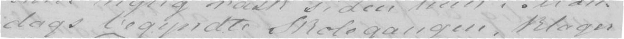dags begyndte Skolegangen, klager
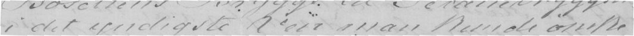i det yndigste Veir man kunde ønske
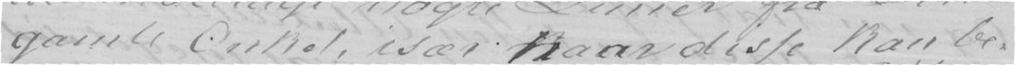gamle Onkel, især naar disse kan be-
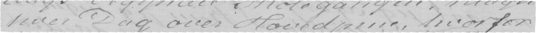hver Dag over Hovedpine, hvorfor
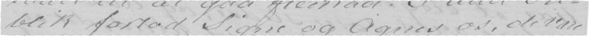blik forlod Signe og Agnes os, de vare
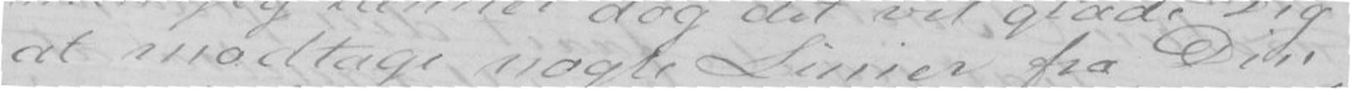at modtage nogle Linier fra Din
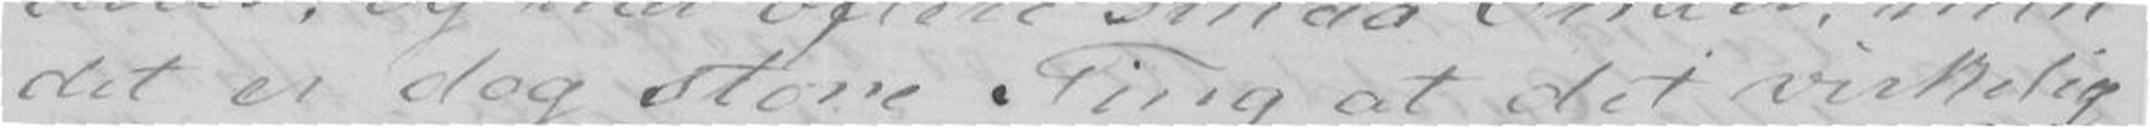det er dog store Ting at det virkelig
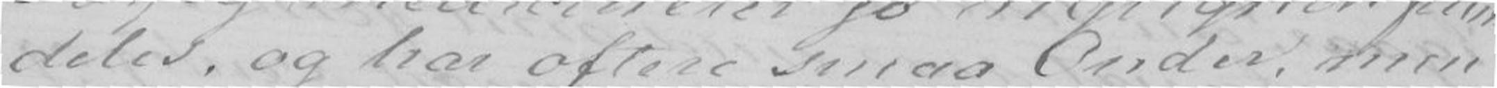deles, og har oftere smaa Onder, men
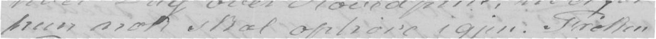hun nok skal ophøre igjen. Frøken
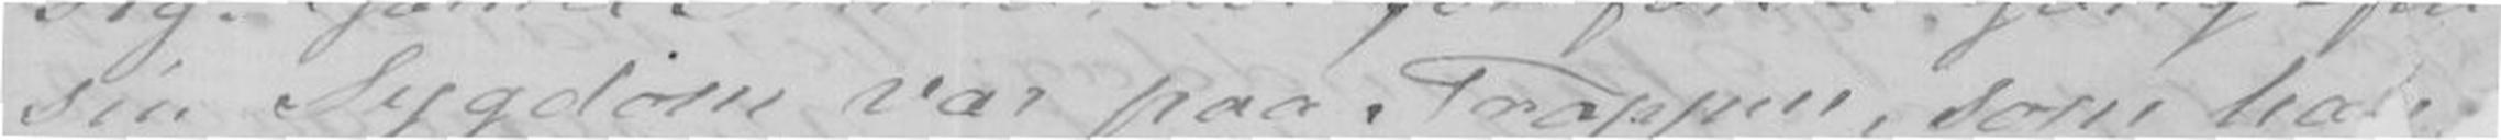sin Sygdom var paa Trappen, som ha
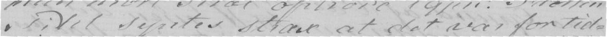Pihl syntes stax at det var for tid-
